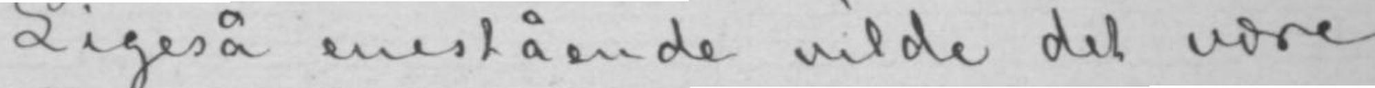Ligeså enestående vilde det være
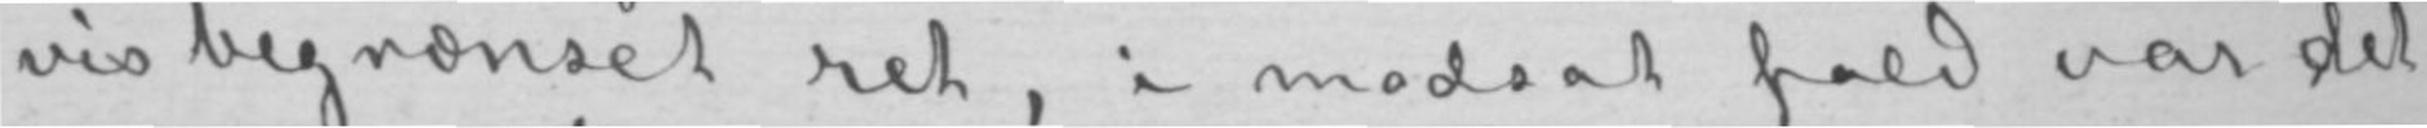vis begrænset ret, i modsat fald var det
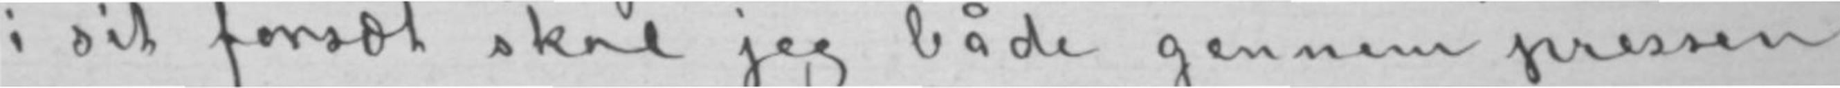i sit forsæt skal jeg både gennem pressen
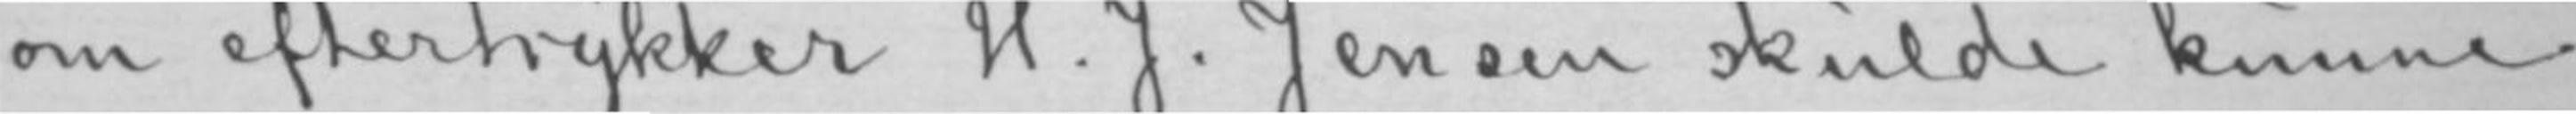om eftertrykker H. J. Jensen skulde kunne
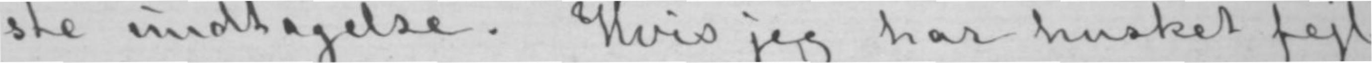ste undtagelse. Hvis jeg har husket fejl
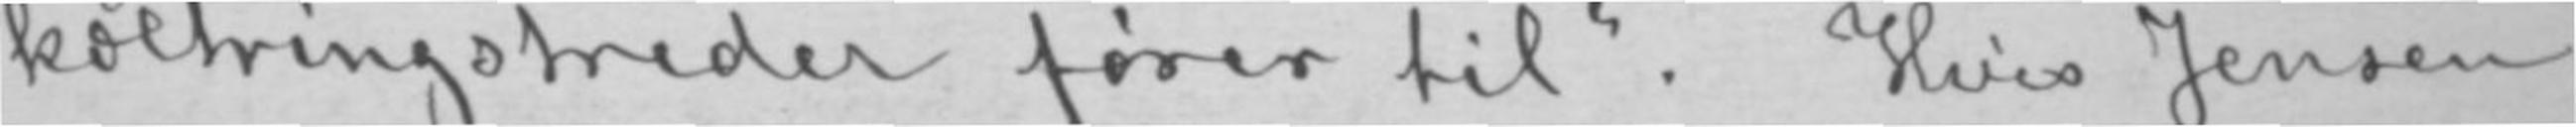kæltringstreder fører til». Hvis Jensen
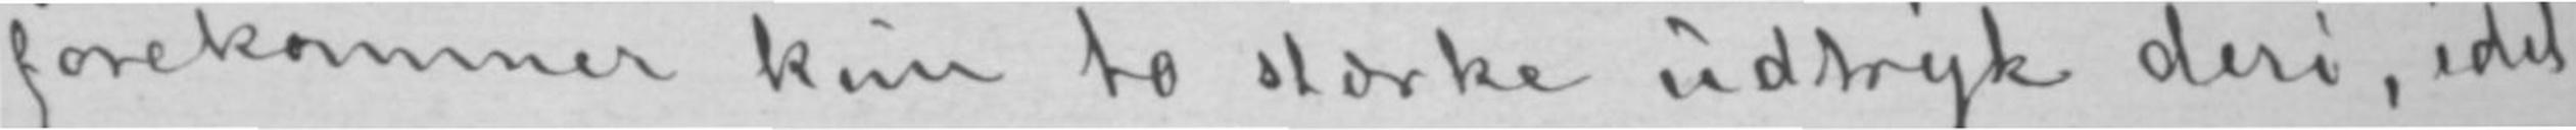forekommer kun to stærke udtryk deri, idet
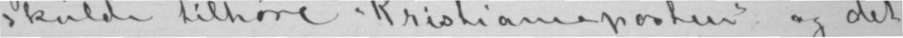skulde tilhøre «Kristianiaposten» og det
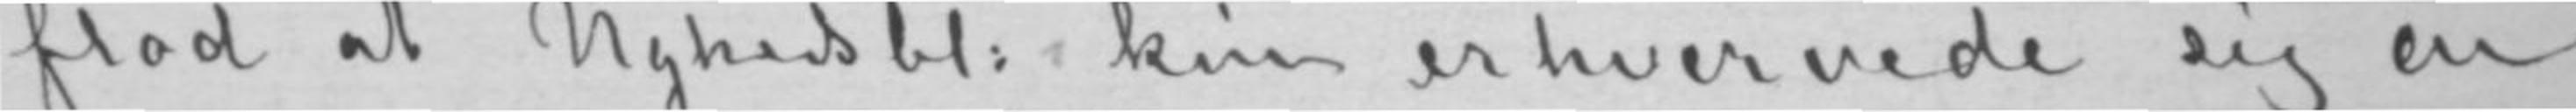flod at Nyhedsbl: kun erhvervede sig en
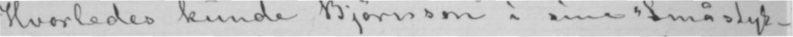Hvorledes kunde Bjørnson i sine «Småstyk-
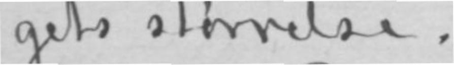gets størrelse.
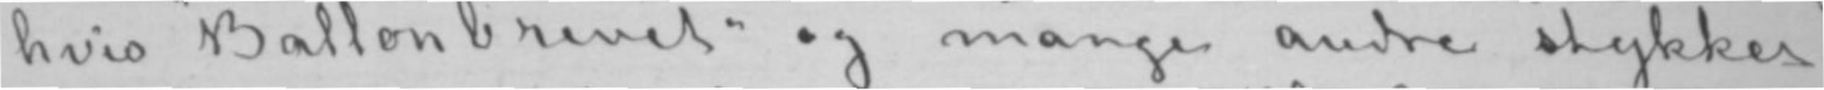hvis «Ballonbrevet» og mange andre stykker
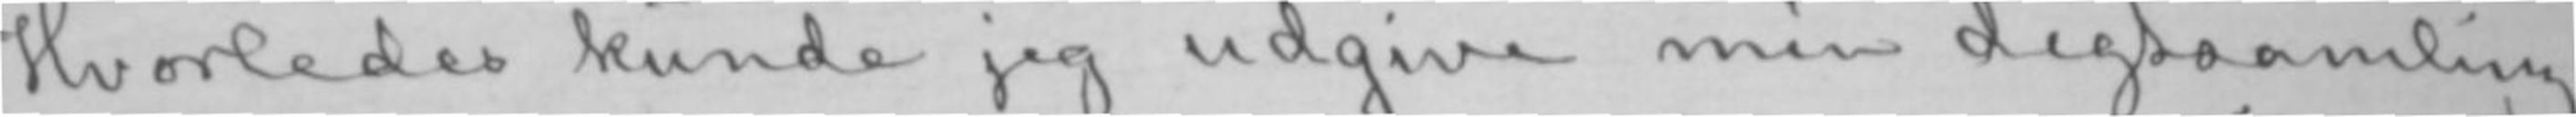Hvorledes kunde jeg udgive min digtsamling
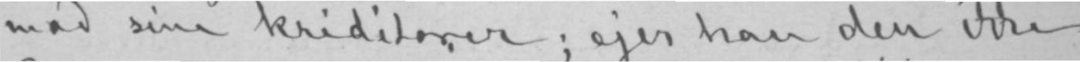mod sine kreditorer; ejer han den ikke
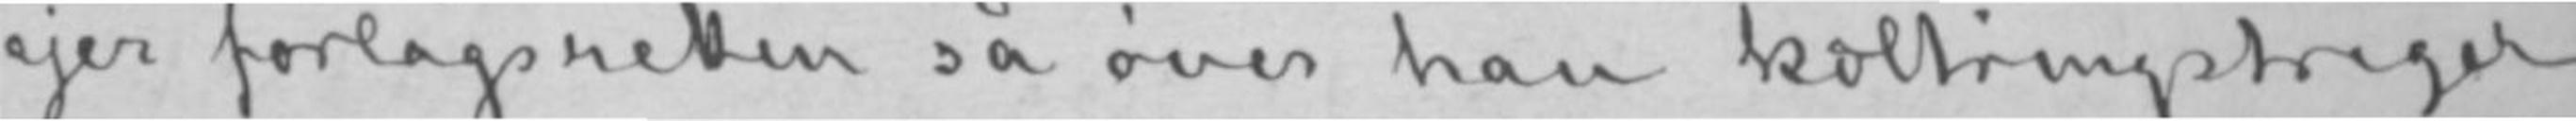ejer forlagsretten så øver han kæltringstreger
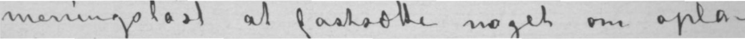meningsløst at fastsætte noget om opla-
